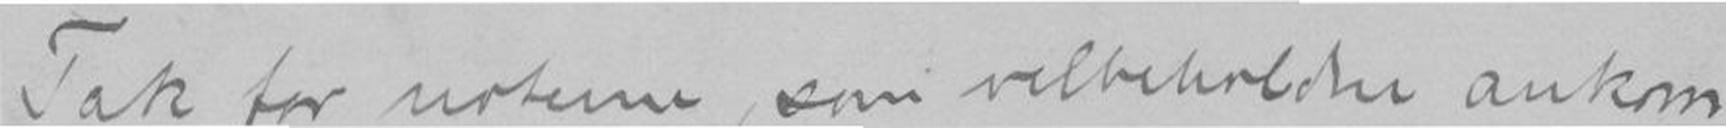Takk for noterne, som velbeholden ankom
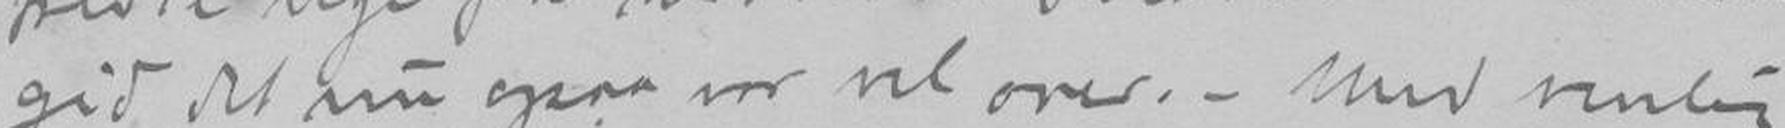gid det nu ogsaa var vel over.- Med venlig
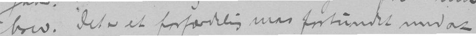brev. Det er et forfærdelig mas forbundet med at
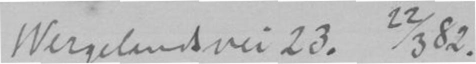Wergelandsvei 23. 22/3 82.
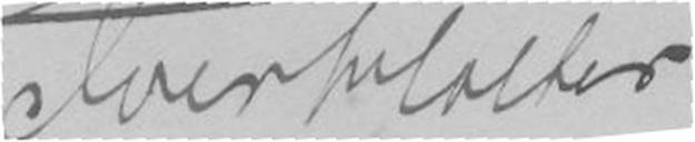Iver Holter
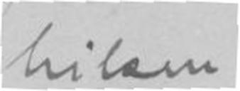hilsen
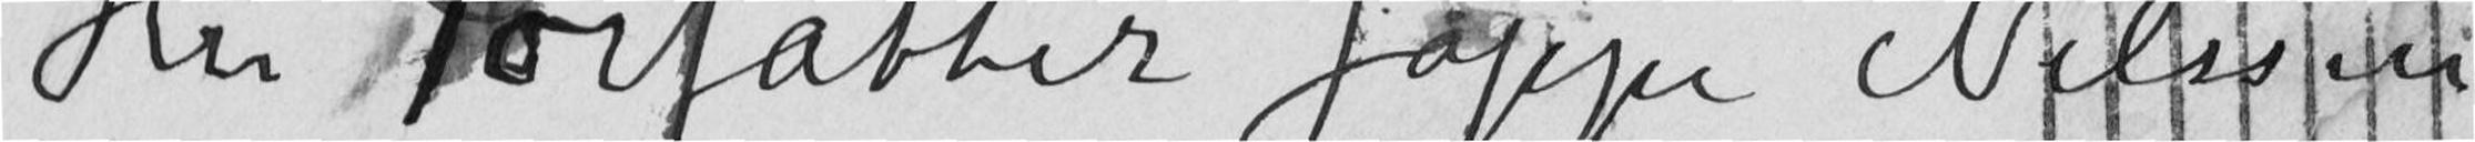Hrr Forfatter Jappe Nilssen
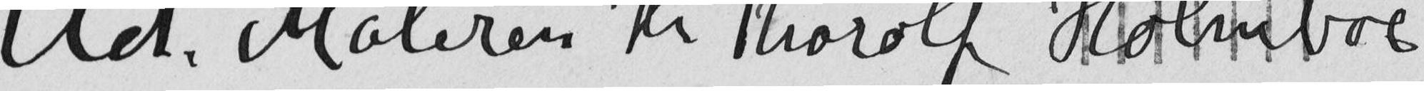Adr. Maleren Hr Thorolf Holmboe
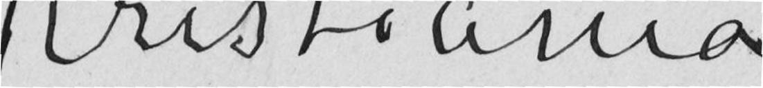Kristiania
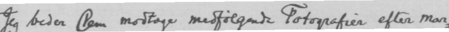Jeg beder Dem modtage medfølgende Fotografier efter mars
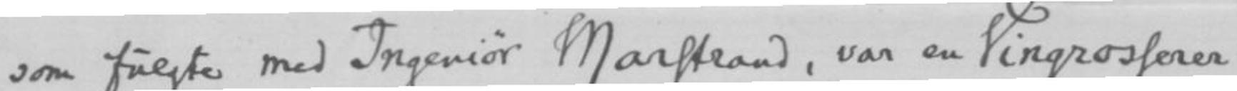som fulgte med Ingeniør Marstrand, var en Vingroserer
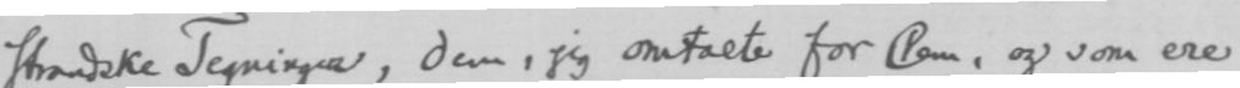trandske Tegninger, dem, jeg omtalte for Dem, og som ere
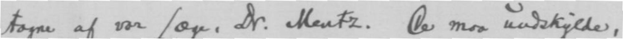tagne af vor Læge. Dr. Mentz. De maa undskylde
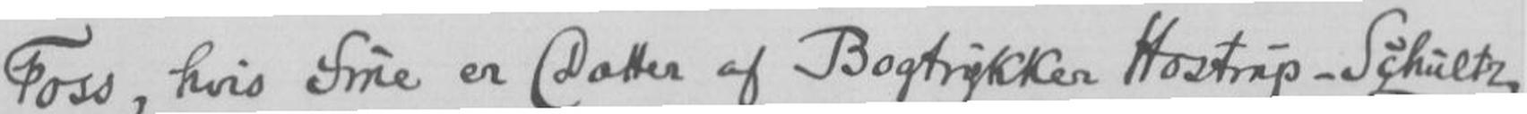Foss, hvis Frue er Datter af Bogtrykker Hostryp-Schultz.
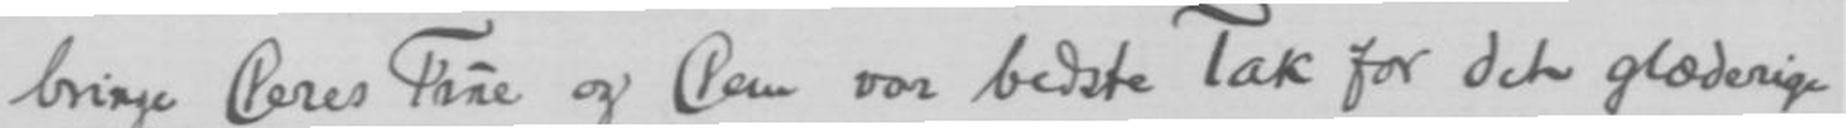bringe Deres Frue og Dem vor bedste Tak for det glædelige
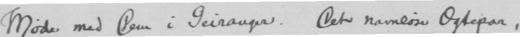Møde med Dem i Geiranger. Det navnløse Ægtepar,
